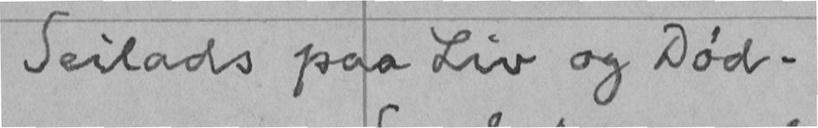Seilads paa Liv og Død.
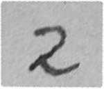2
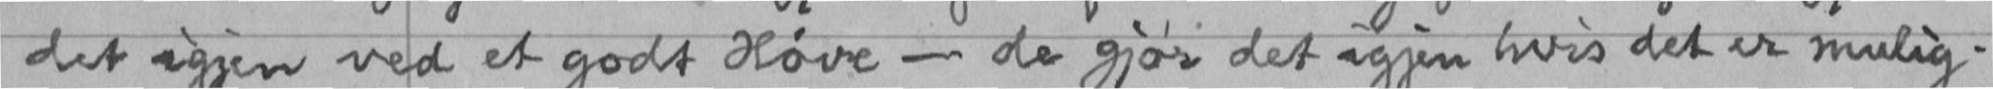det igjen ved et godt Høve - de gjør det igjen hvis det er mulig.
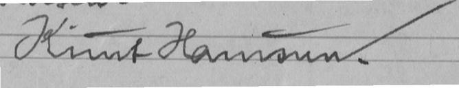Knut Hamsun.
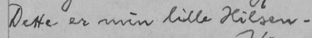Dette er min lille Hilsen.
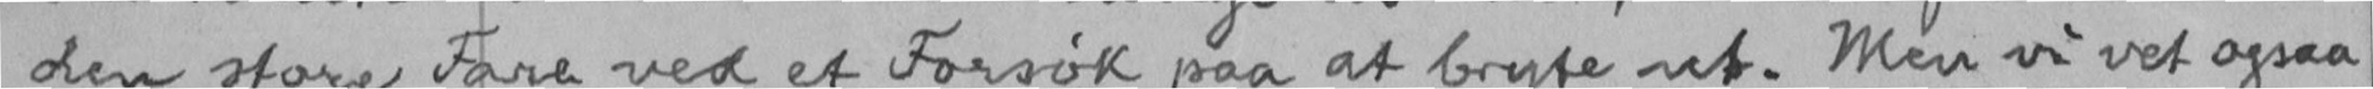den store Fare ved et Forsøk paa at bryte ut. Men vi vet ogsaa
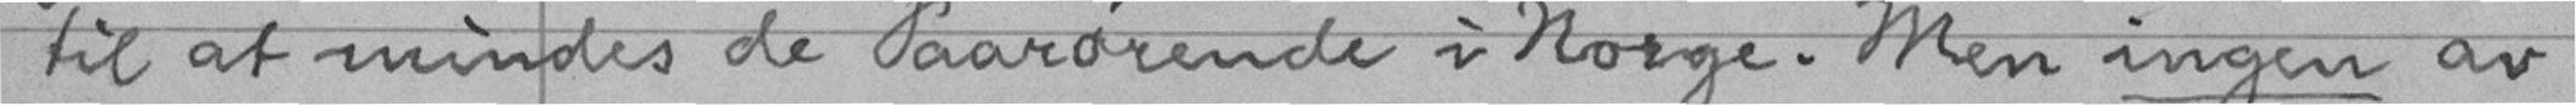til at mindes de Paarørende i Norge. Men ingen av
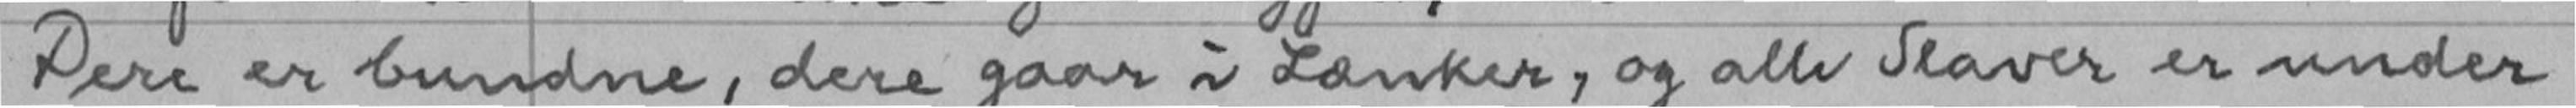Dere er bundne, dere gaar i Lænker, og alle Slaver er under
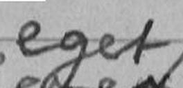eget
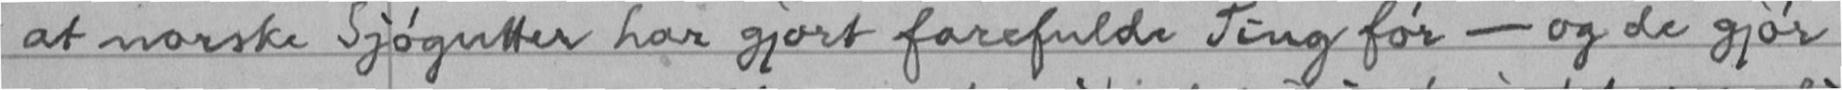at norske Sjøgutter har gjort farefulde Ting før - og de gjør
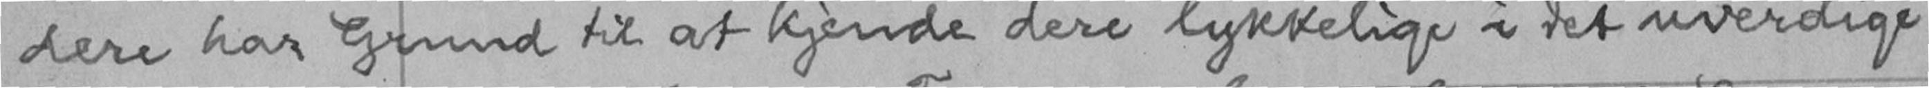dere har Grund til at kjende dere lykkelige i det uverdige
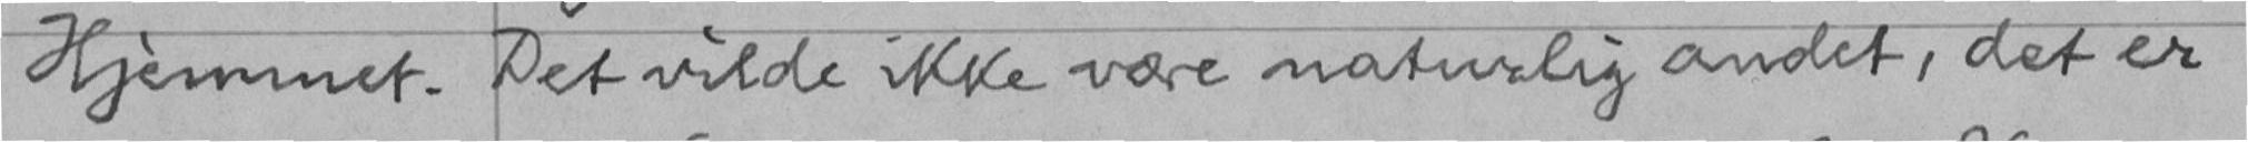Hjemmet. Det vilde ikke være naturlig andet, det er
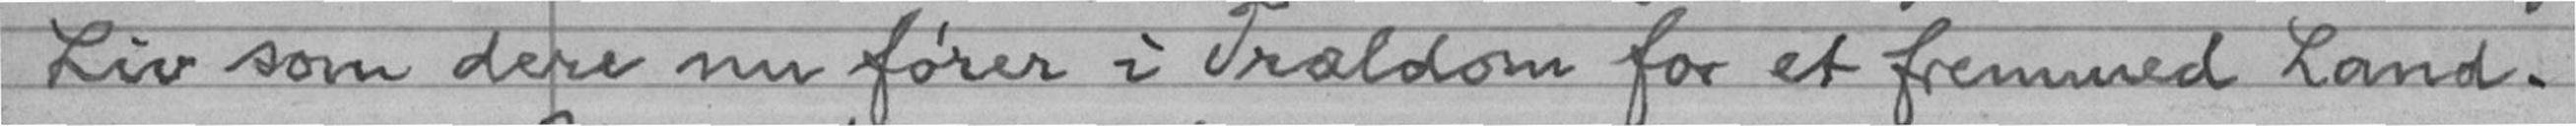Liv som dere nu fører i Trældom for et fremmed Land.
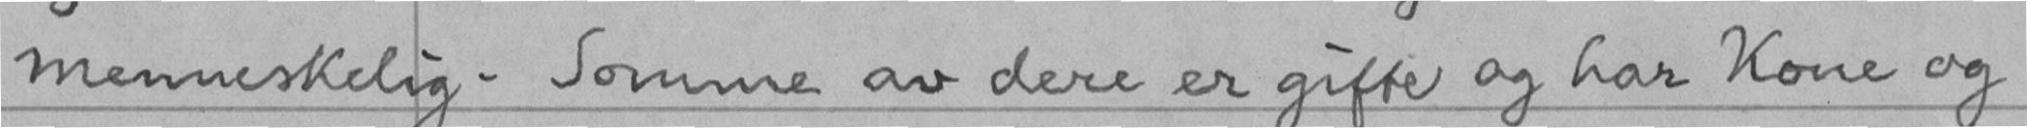menneskelig. Somme av dere er gifte og har Kone og
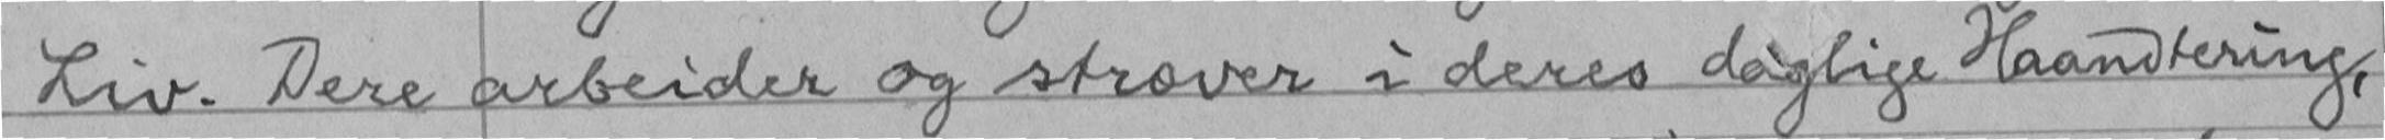Liv. Dere arbeider og stræver i deres daglige Haandtering,
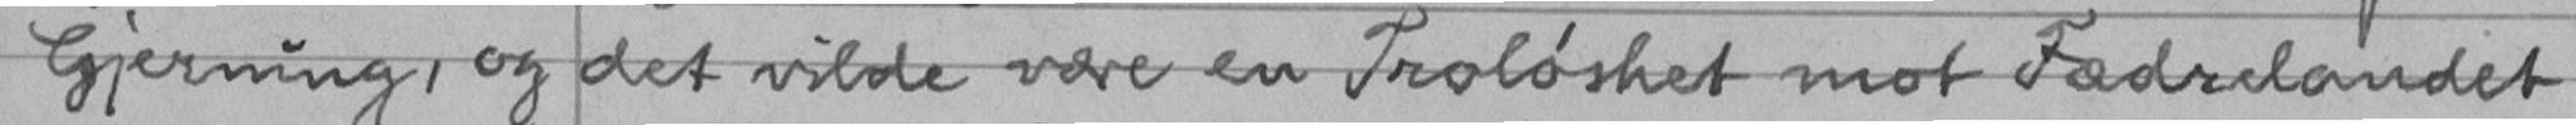Gjerning, og det vilde være en Troløshet mot Fædrelandet
